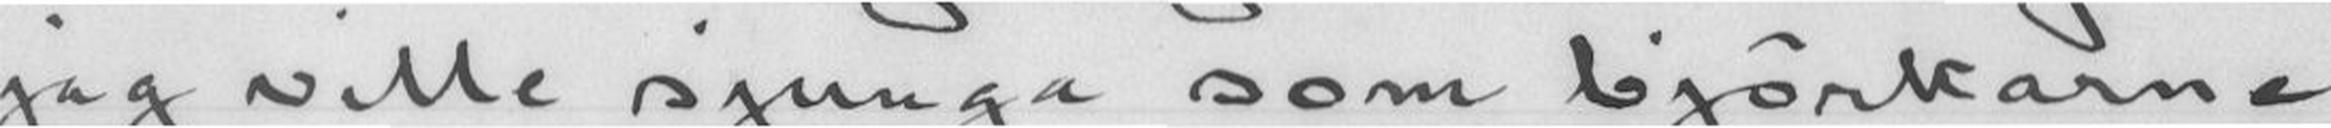jag ville sjunga som björkarne
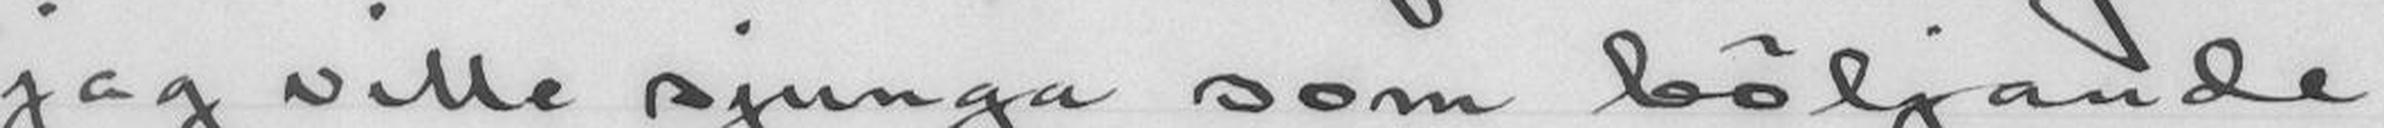jag ville sjunga som böljande
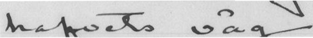hafvets våg
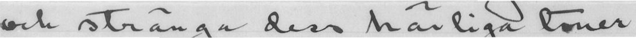och stränga dess härliga toner
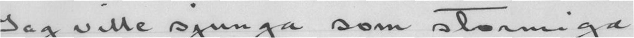Jag ville sjunga som stormiga
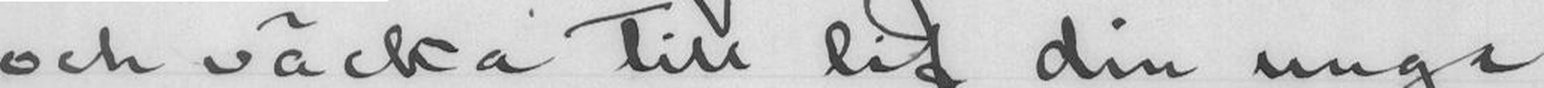och väcka till lif din unga
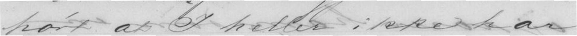hørt at I heller ikke har
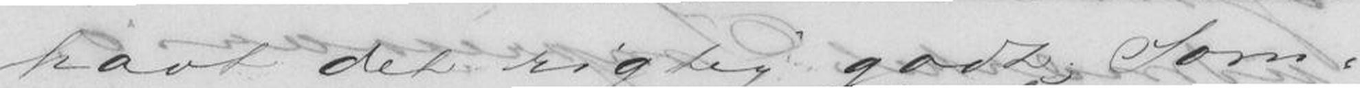havt det rigtig godt, Som-
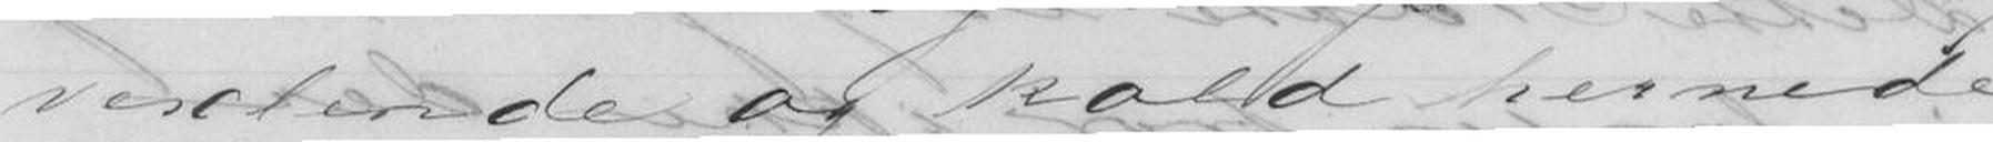vexlende og kold hernede,
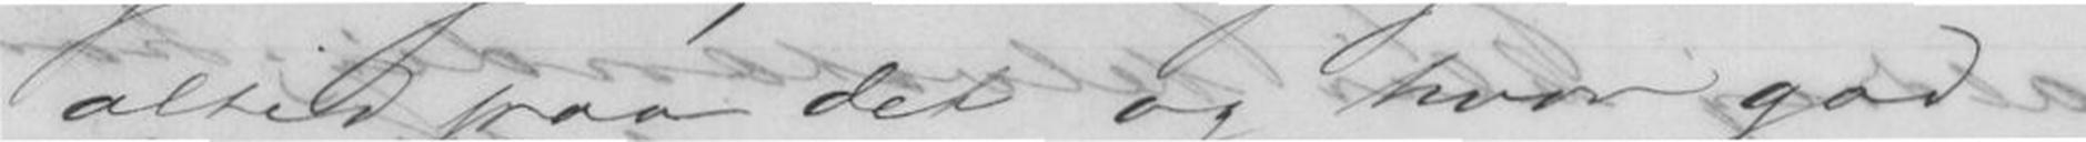altid paa det og hvor god
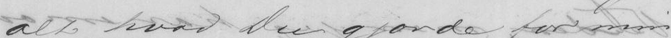alt hvad Du gjorde for min
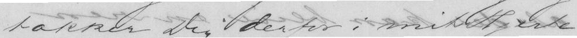takker Dig derfor i mit Hjerte.
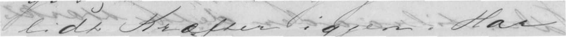lidt Kræfter igjen. Har
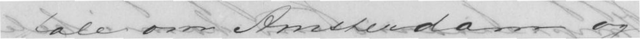tale om Amsterdam og
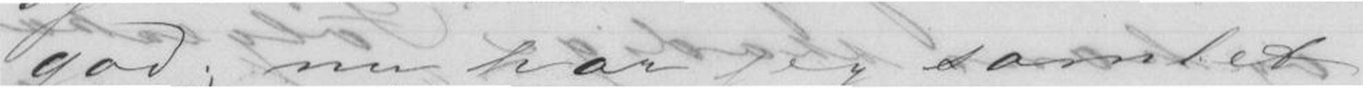god; nu har jeg samlet
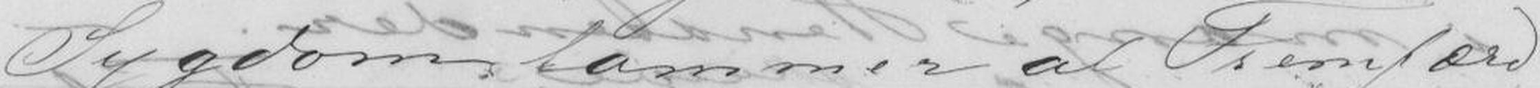Sygdom lammer al Fremfærd,
